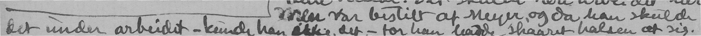det under arbeidet - kunde han ikke det - for han havde skaaret halsen at sig.
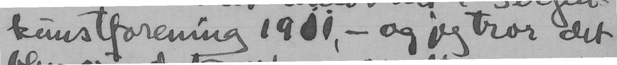kunstforening 19111, - og jeg tror det
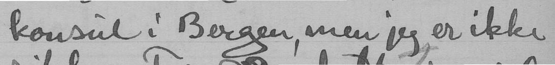konsul i Bergen, men jeg er ikke
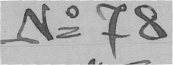No 78
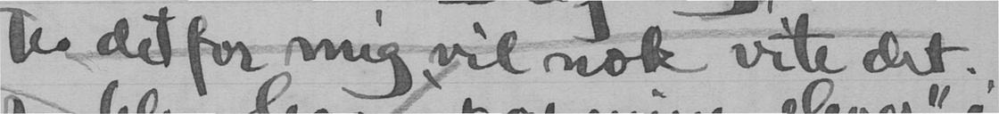te det for mig vil nok vite det.
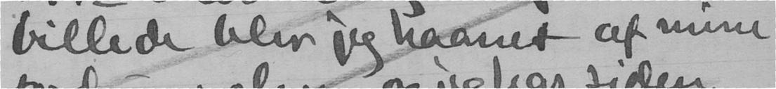billede blev jeg haanet af mine
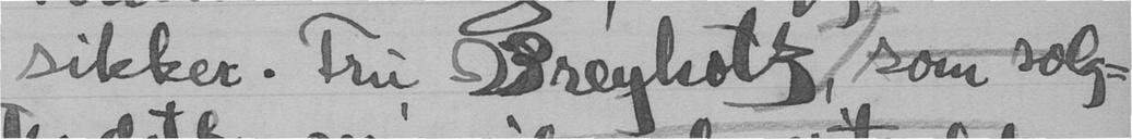sikker. Fru Breyholtz, som solg-
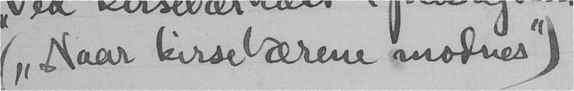("Naar kirsebærene modnes")
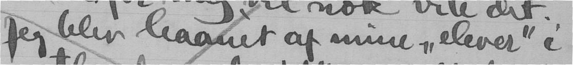Jeg blev haanet af mine "elever" i
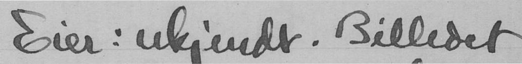Eier: ukjendt. Billedet
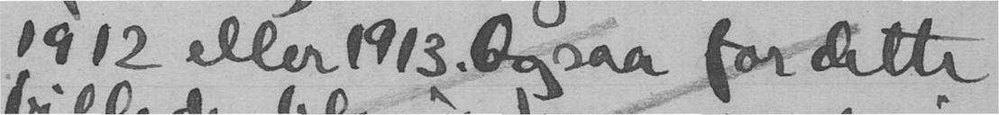1912 eller 1913 ogsaa for dette
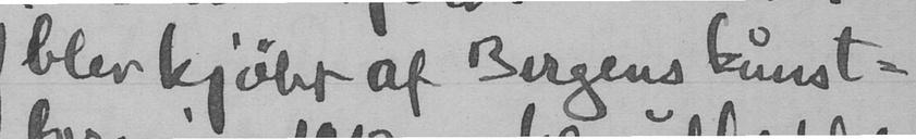blev kjøbt af Bergens kunst-
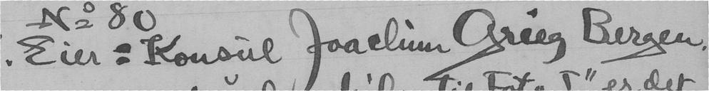Eier: Konsul Joachim Greig Bergen,
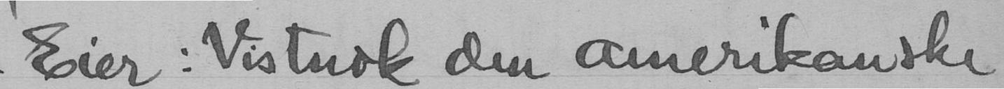Eier: Vistnok den amerikanske
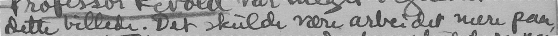dette billede. Dat skulde være arbeidet mere paa,
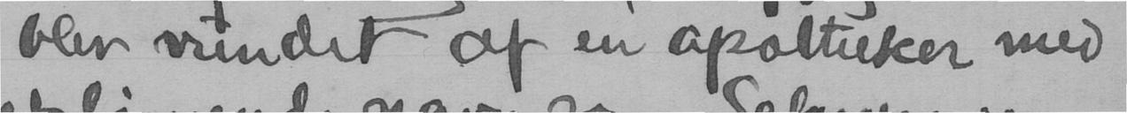blev vundet af en apotheker med
